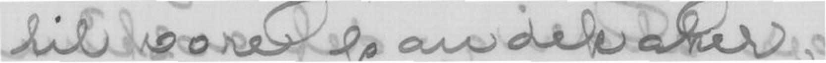til vore pandekaker,
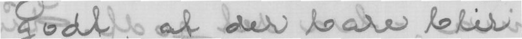godt, at der bare blir
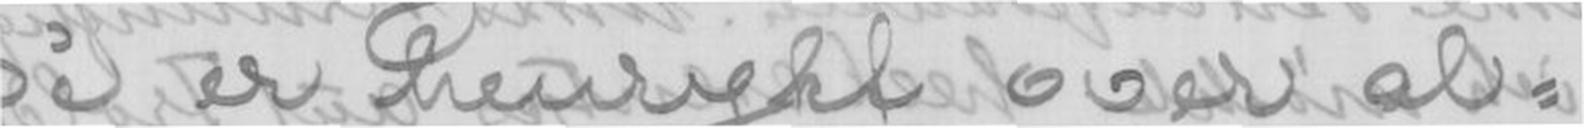Dé er henrykt over al-
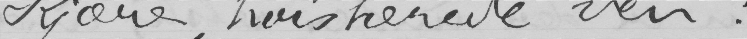Kjære, høistærede ven!
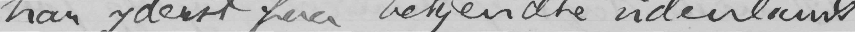har yderst faa bekjendte udenlands
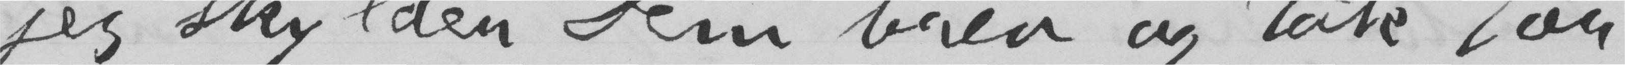jeg skylder Dem brev og tak for
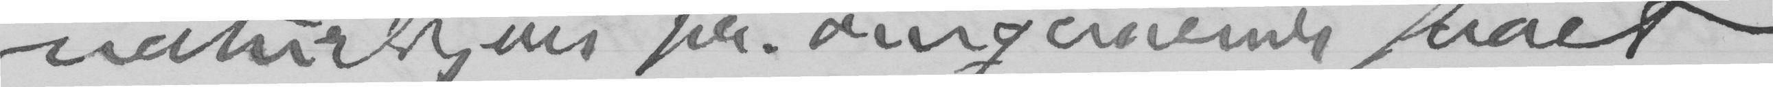naturligvis pr. omgaaende faaet
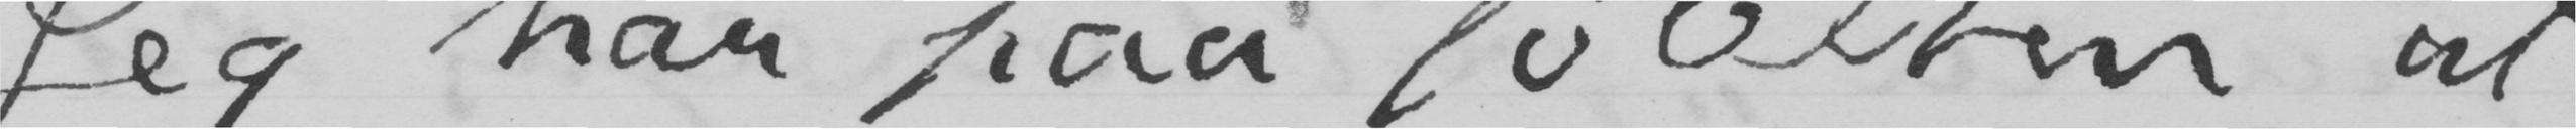Jeg har paa følelsen, at
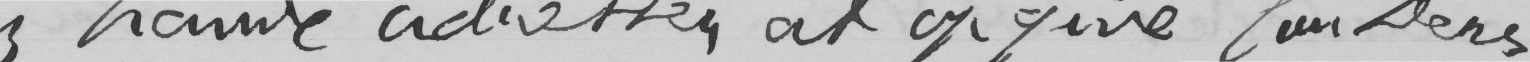jeg havde adresser at opgive for Deres
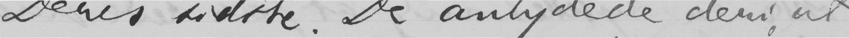Deres sidste. De antydede deri at
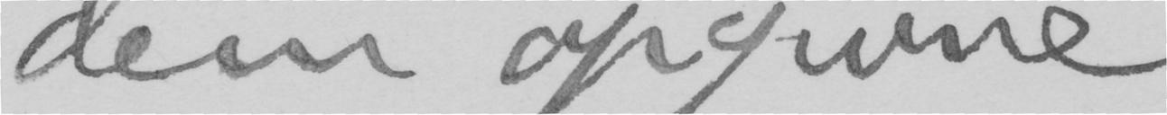dem opgivne.
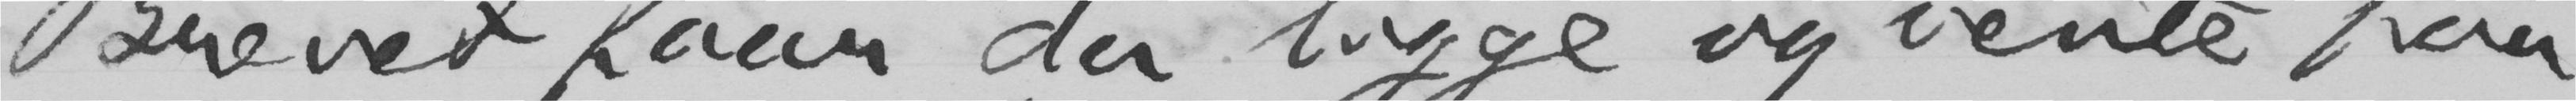Brevet faar da ligge og vente paa
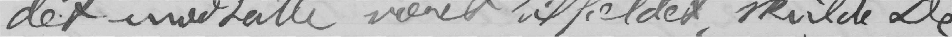det modsatte været tilfælde, skulde De
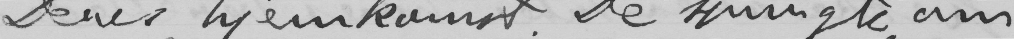Deres hjemkomst. De spurgte om
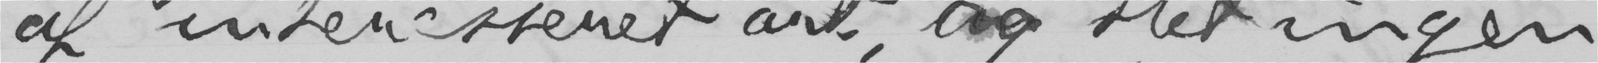af interesseret art, og slet ingen
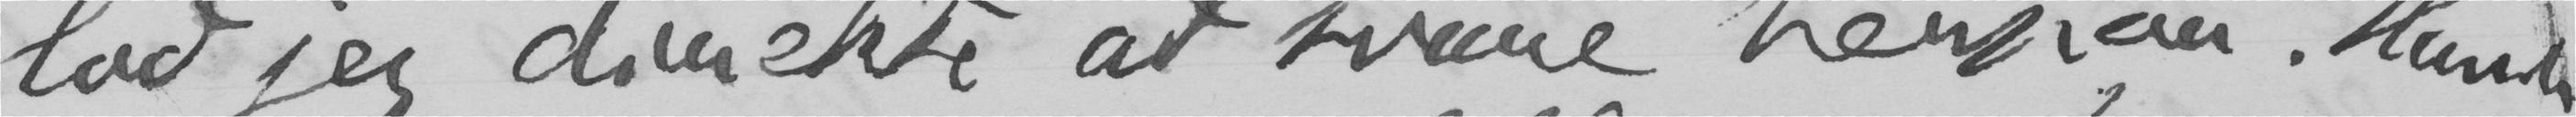lod jeg direkte at svare herpaa. Havde
